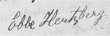Ebbe Hertzberg
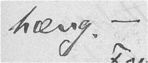hæng. -
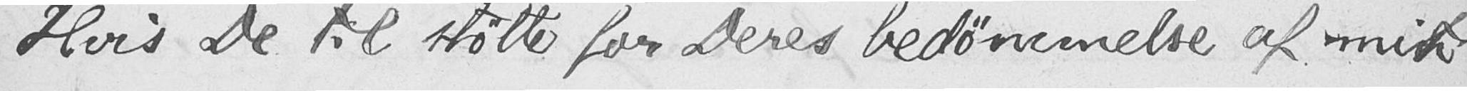Hvis De til støtte for Deres bedømmelse af mit
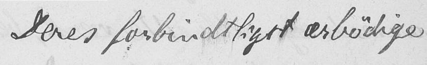Deres forbindtligst ærbødige
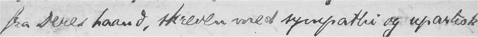fra Deres haand, skreven med sympathi og upartisk
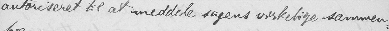autoriseret til at meddele sagens virkelige sammen-
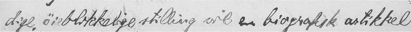dige, øieblikkelige stilling vil en biografisk artikkel
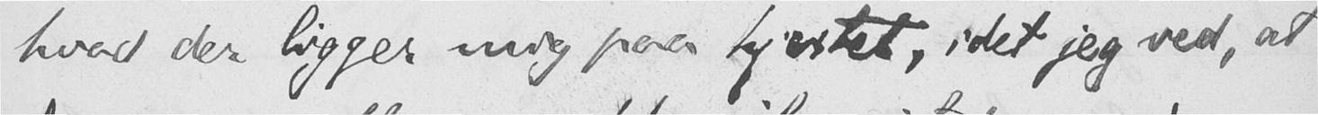hvad der ligger mig paa hjertet, idet jeg ved, at
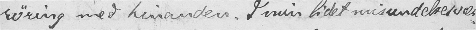røring med hinanden. I min lidet misundelsesvær-
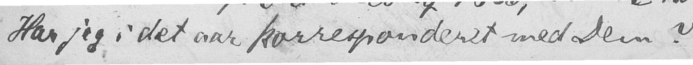Har jeg i det aar korresponderet med Dem?
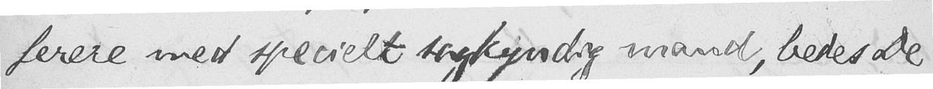ferere med specielt sagkyndig mand, bedes De
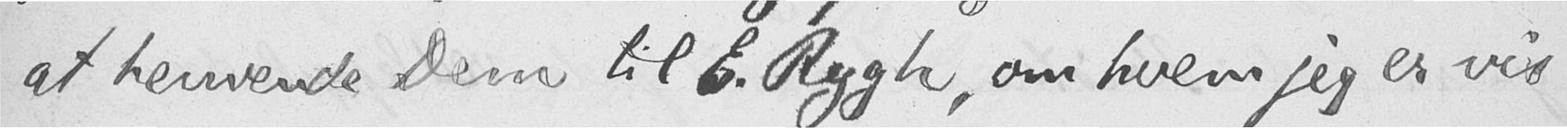at henvende Dem til E. Rygh, om hvem jeg er vis
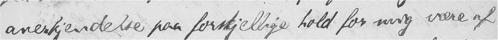anerkjendelse paa forskjellige hold for mig være af
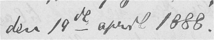den 19de april 1888.
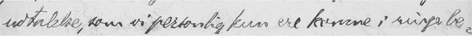udtalelse, som vi personlig kun ere komne i ringe be-
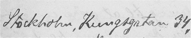Stockholm, Kungsgatan 34,
